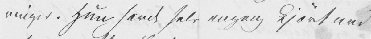vinger. Hun havde selv engang kjørt ned
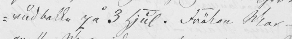rudbakke på 3 Hjul. Frøken Mar-
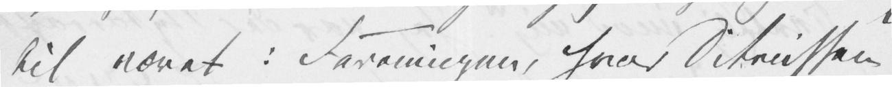til været i Foreningen, hvor Ditrichson
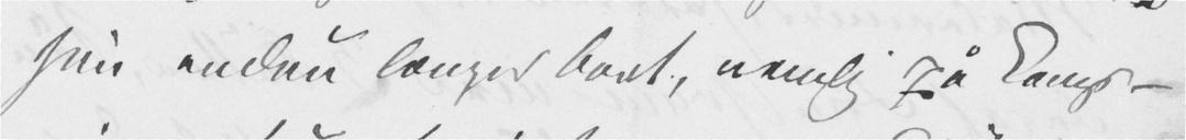hun endnu længer bort, nemlig på Kongs-
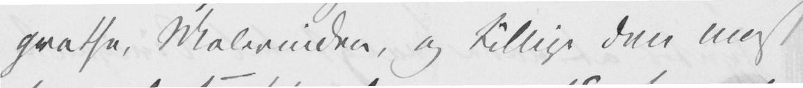grethe, Malerinden, og tillige den mest
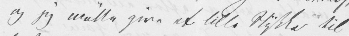og jeg måtte give et lille Stykke til
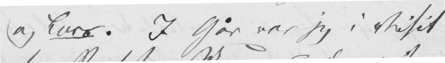og Lars. I Går var jeg i Visit
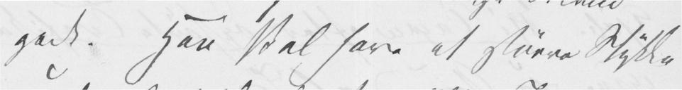godt. Han skal have et større Stykke
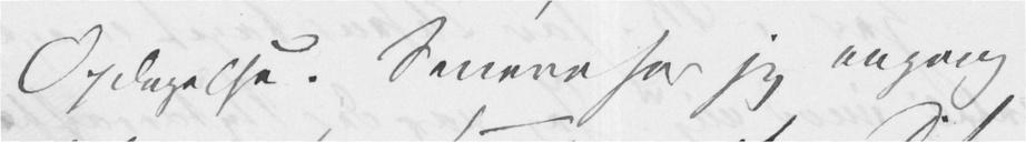Opdagelse. Senere har jeg engang
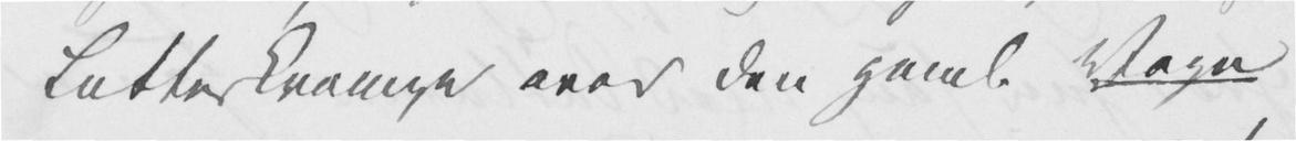Latterkrampe over den gamle Vogn
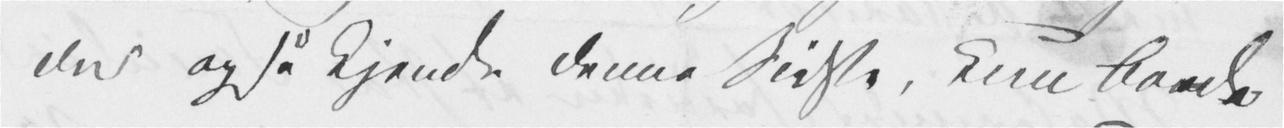der også kjendte denne Sidste, kun boede
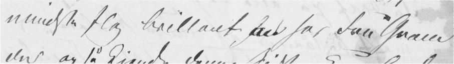mindste slog brillant an hos Fru Gram
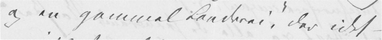og en gammel Landevei," der idet-
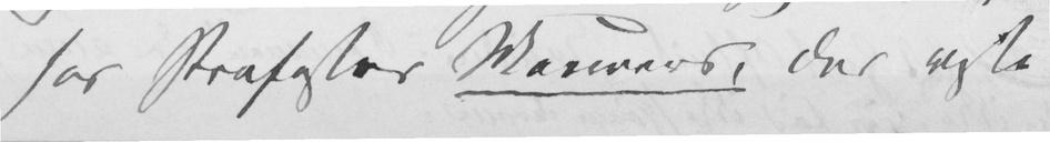hos Professor Maurers, der viste
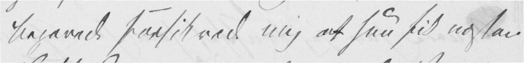begavede forsikrede mig at hun fik næsten
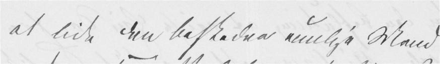at lide den beskedne umulige Mand
